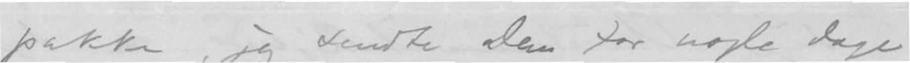pakke, jeg sendte Dem for nogle dage
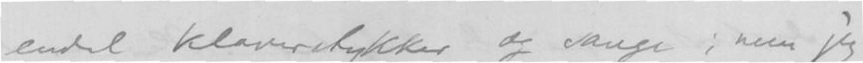endel klaverstykker og sange; men jeg
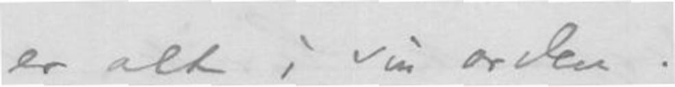er alt i sin orden.
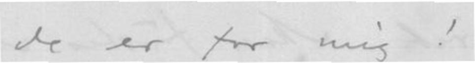de er for mig!
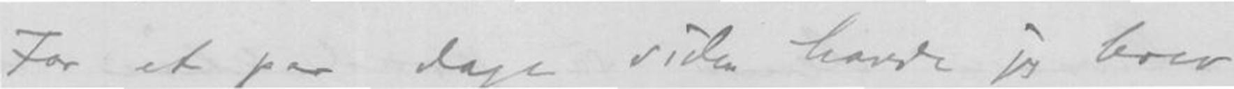For et par dage siden havde jeg brev
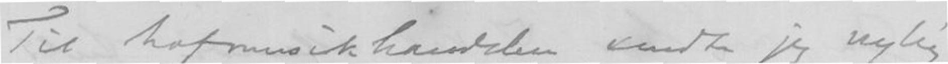Til hofmusikhandelen sendte jeg nylig
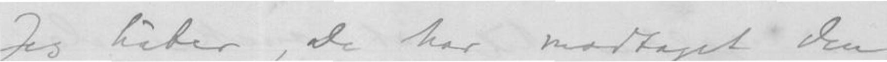Jeg håber, De har modtaget den
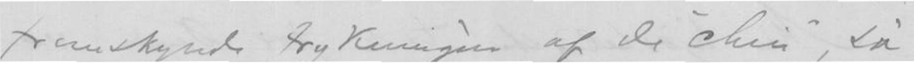fremskynde trykningen af de "chin.", så
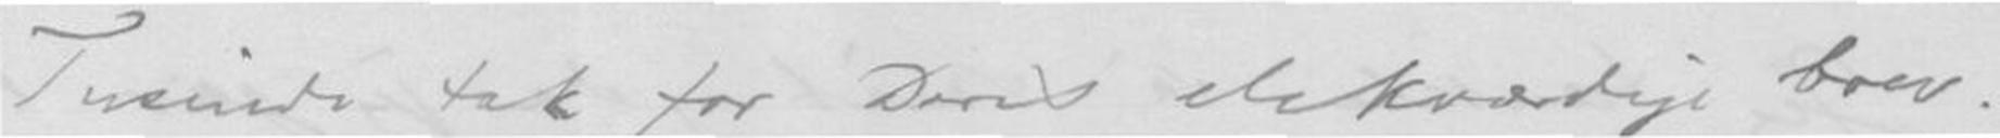Tusind tak for Deres elskværdige brev.
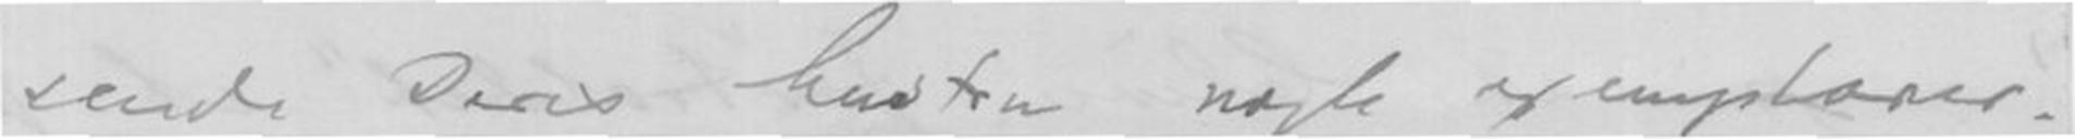sende Deres hustru nogle exsemplarer.
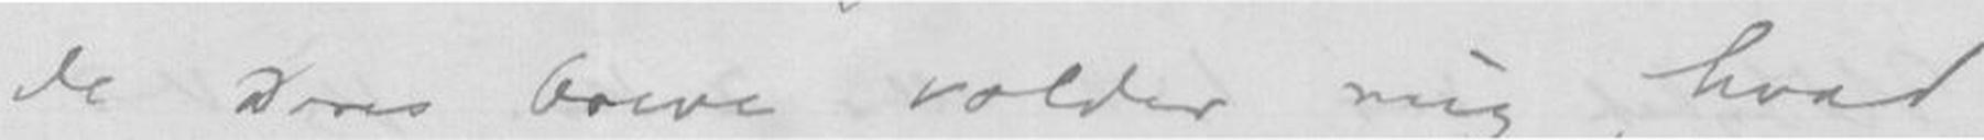de Deres breve volder mig, hvad
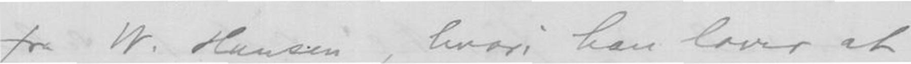fra W. Hansen, hvori han lover at
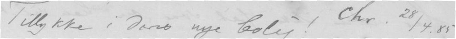Tillykke i Deres nye bolig!Chr. 28/4.85
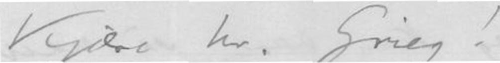Kjære hr. Grieg!
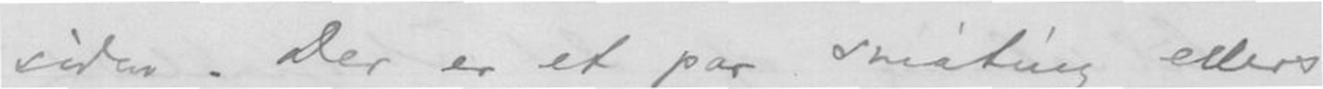siden. Der er at par småting ellers
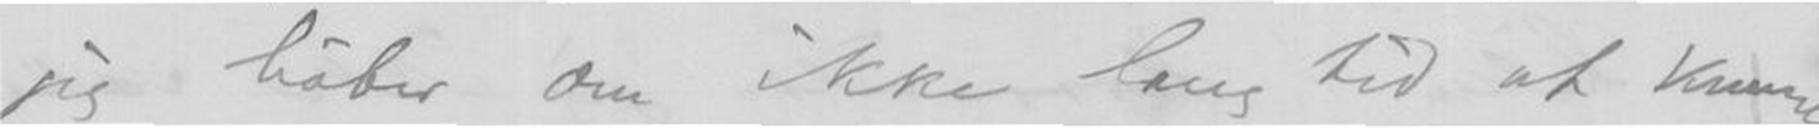jeg håber om ikke lang tid at kunne
